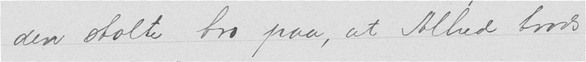den stolte tro paa, at Alhed trods
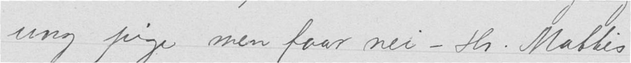ung pige men faar nei – Hr. Mathis
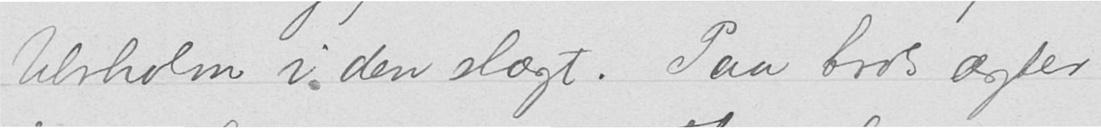Ulvholm i den slægt. Paa trods ægter
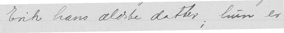Erik hans ældste datter, hun er
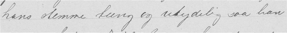hans stemme tung og utydelig saa han
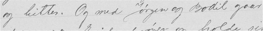og bitter. Og med Jørgen og Bodil gaar
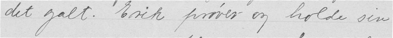det galt. Erik prøver og holde sin
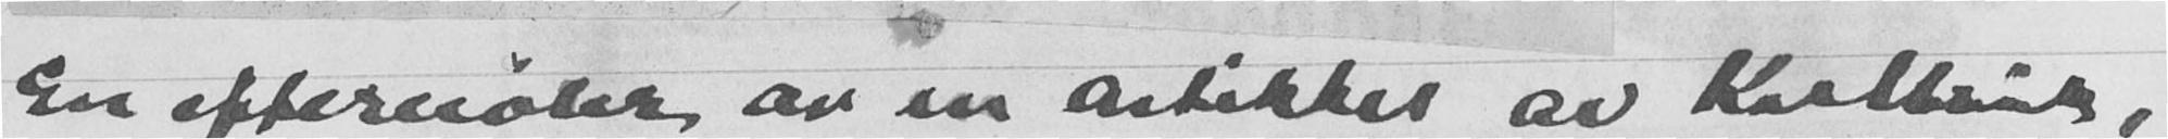En eftersøkt, av en artikkel av Kasttis,
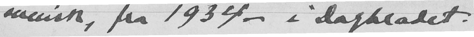svensk, fra 1934 - i Dagbladet.
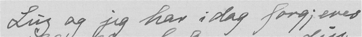Luz og jeg har i dag forgjeves
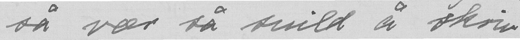så vær så snild å skriv
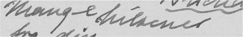Mange hilsener
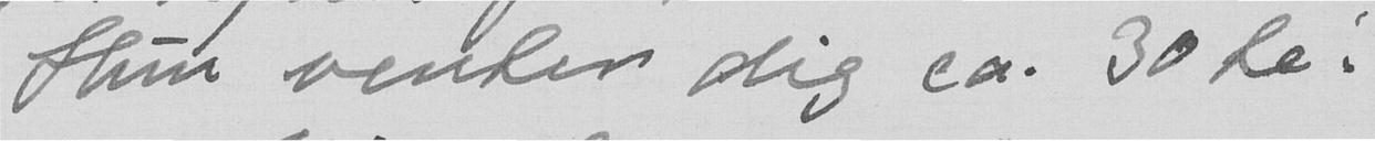Hun venter dig ca. 30te.
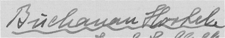Buchanan Hostel
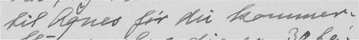til Agnes før du kommer.
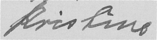Kristine
